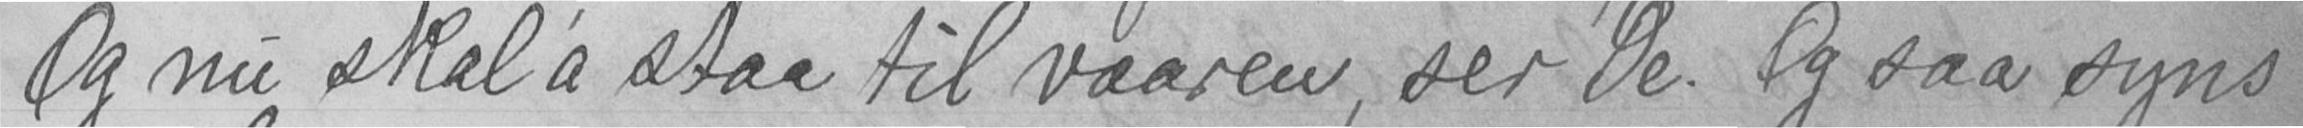Og nu skal'a staa til vaaren, ser De. Og saa syns
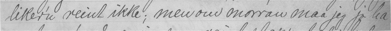liker'n reint ikke; men om morran maa jeg jo ha
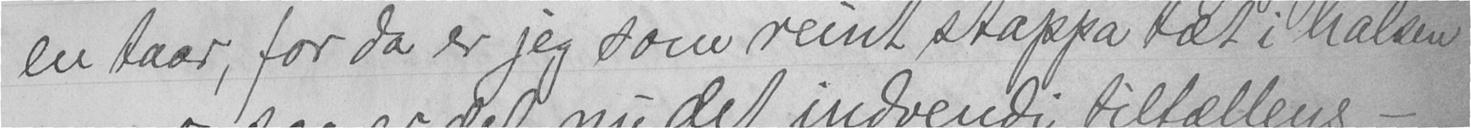en taar, for da er jeg som reint stappa tæt i halsen
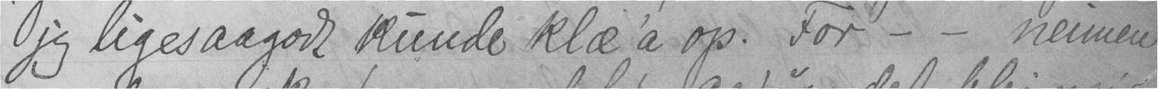jeg ligesaagodt kunde klæ'a op. For - - neimen
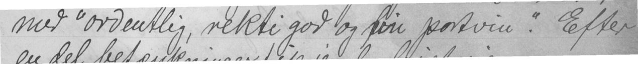med "ordentlig, rekti god og fin portvin." Efter
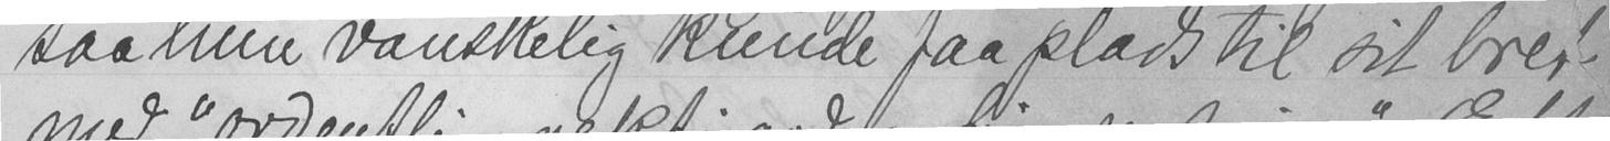saa hun vanskelig kunde faa plads til sit bret
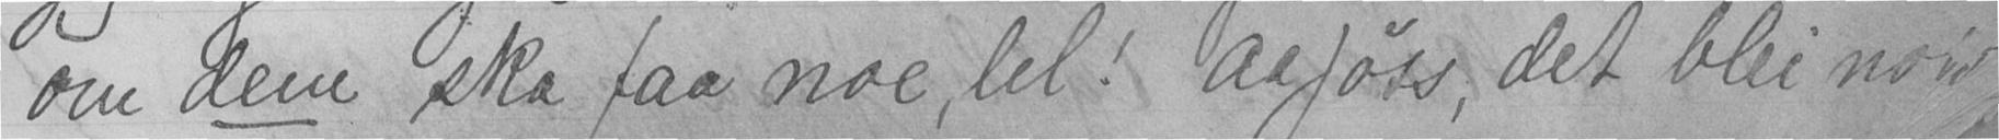om dem ska faa noe, lel! aa jøss, det blei nok
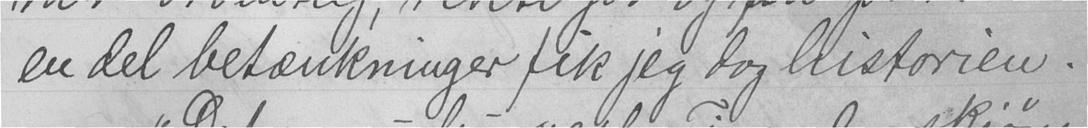en del betænkninger fik jeg dog historien.
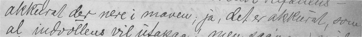akkurat der nere i maven; ja, det er akkurat, som
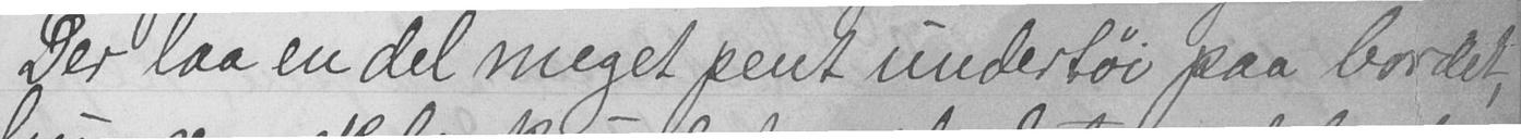Der laa en del meget pent undertøi paa bordet,
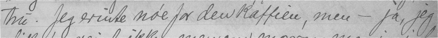tru. Jeg erinte no'e for den kaffien, men - ja, jeg
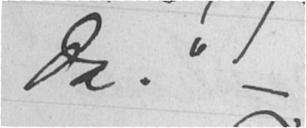da. "
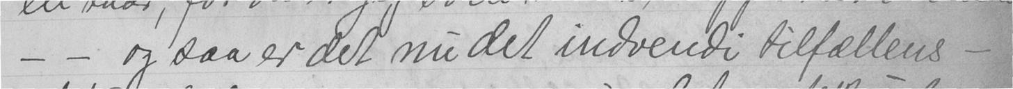- - og saa er det nu det indvendi tilfællens -
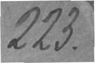223.
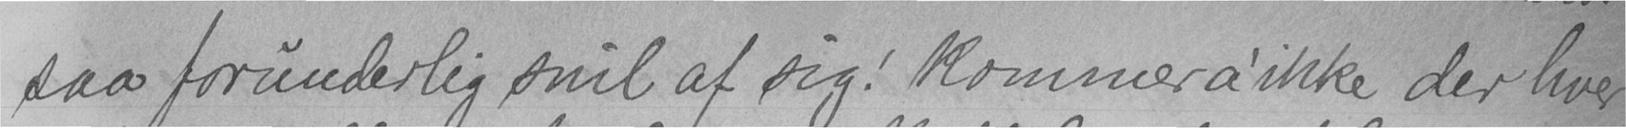saa forunderlig snil af sig! kommer a'ikke der hver
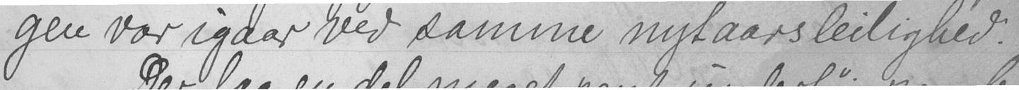gen var igaar ved samme nytaars leilighed.
